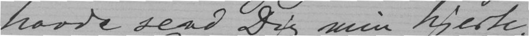havde send Dig min hjerte-
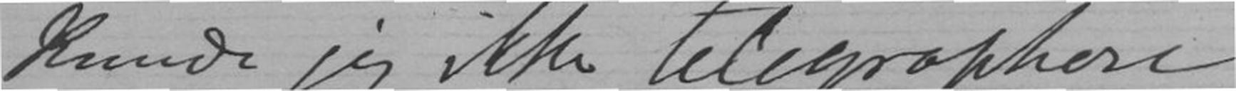kunde jeg ikke telegraphere
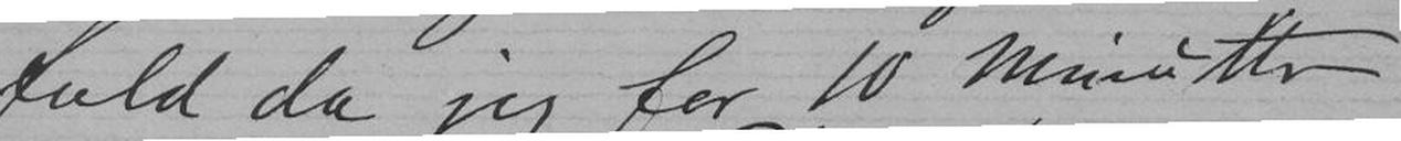fuld da jeg for 10 minutter
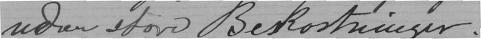uden store Bekostninger.
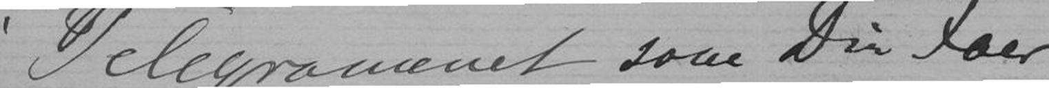i Telegrammet som Din Faer
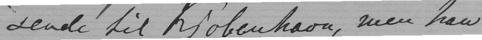sende til Kjøbenhavn, men han
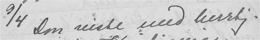9/4 Don reiste med hurtig-
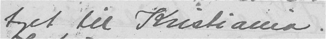toget til Kristiania.
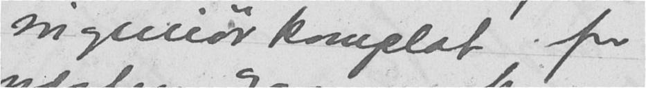ingeniørkomplot for
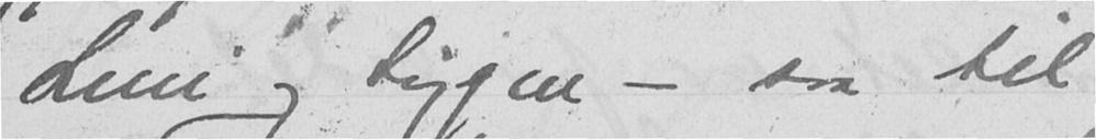Leni og Siggen - saa til
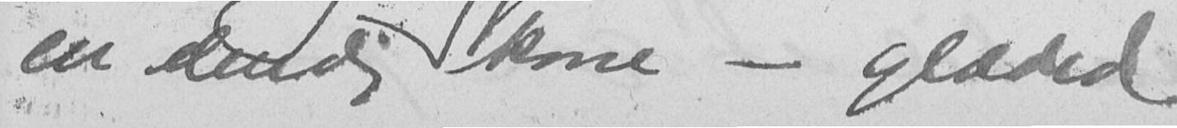en elendig kone - glæded
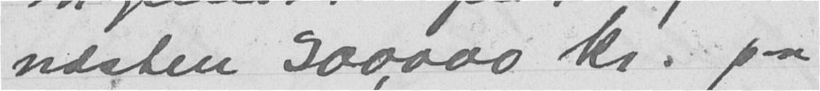næsten 300,000 kr. paa
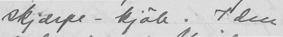skjærpe-kjøb. I den
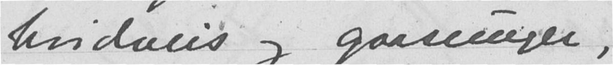hvidveis og gaaseunger,
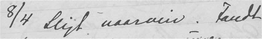8/4 Sligt vaarveir. Fandt
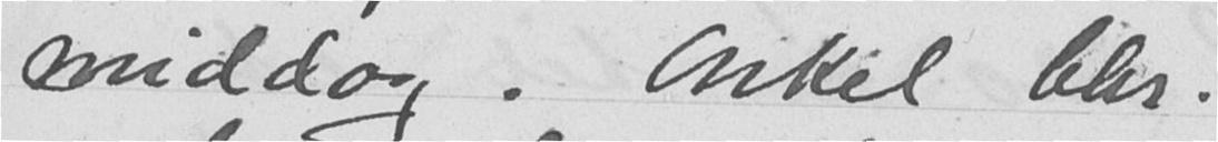middag. Onkel Chr.
